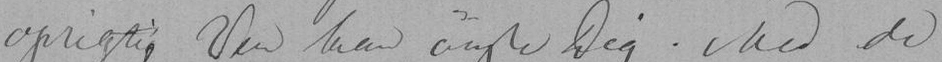oprigtig Ven kan ønske Dig. Med de
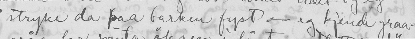stryke da paa barken fyst - eg kjende graa-
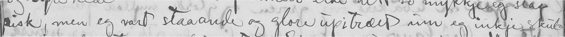pisk, men eg vart staaande og glore upitræet um eg inkje skul-
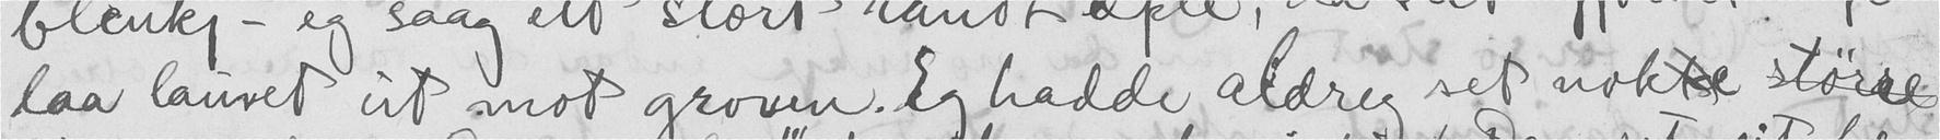laa lauvet at mot groven. Eg hadde aldreg set nok større
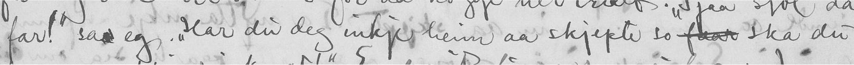far!" saa eg. "Har du deg inkje heim aa skjepte so " Ska du
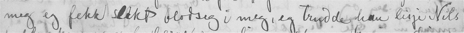meg eg fekk slekt blodseg i meg, eg trydde han lisje Nils
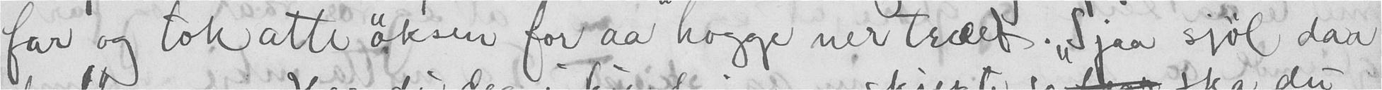far og tok atte øksen for aa hogge ner træet. Sjaa sjøl" daa
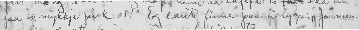faa jo mykkje pisk at!" "Eg laut finne paa ei lygni. "Ja men
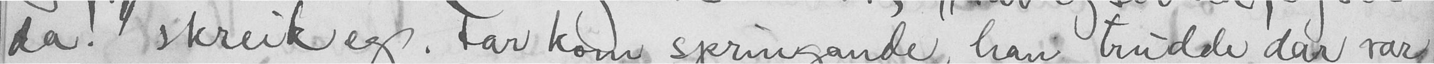da!" skreik eg. Far kom springande, han trude dar var
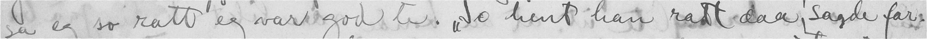sa eg so ratt eg var god te. "So hent hav ratt daa" sagde far.
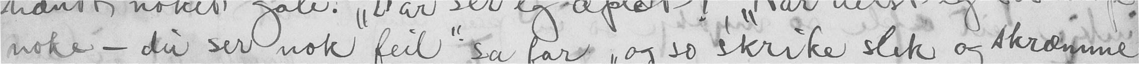noke - du ser nok feil" sa far "og so skrike slek og skræmme
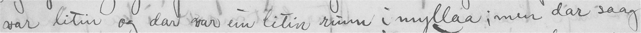var liten og der var ein litin rum i myllaa; men dar saag
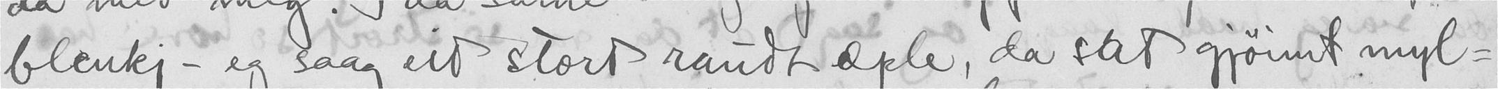blenkj - og saag eit stort raudt æple, da sat gjømt myl-
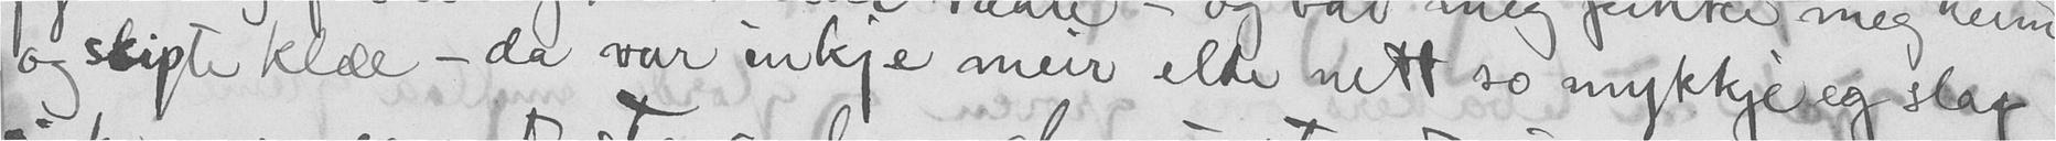og skipte klæe - da var inkje meir elle nettso mykkje eg slap
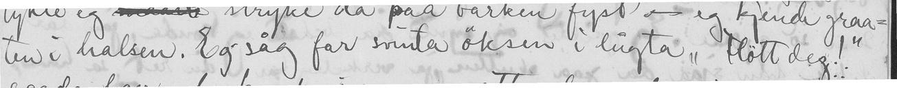ten i halsen. Eg såg far svinta øksen i lugta "fløtt dig!"
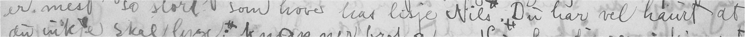er mest so stort som høve har lesje Nils. Du har vel haurt at
